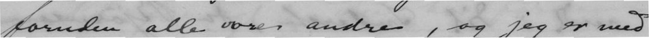foruden alle vore andre, og jeg er med
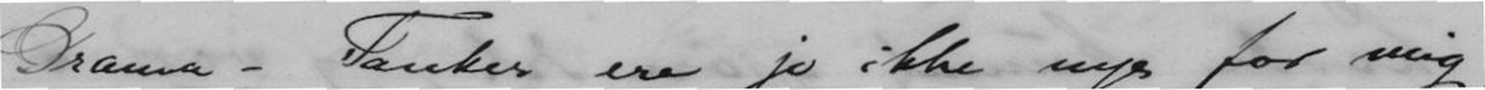Drama-Tanker ere jo ikke nye for mig,
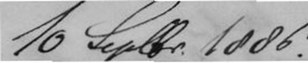10 Septbr. 1886
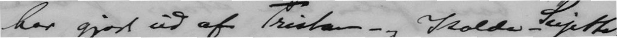har gjort ud af Tristan- og Isolde-Sujettet,
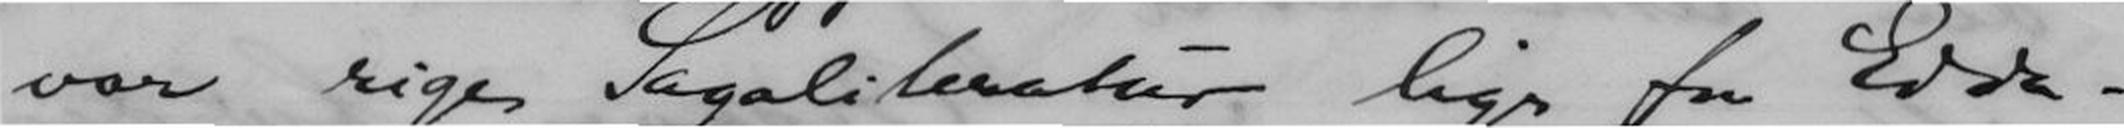vor rige Sagaliteratur lige fra Edda-
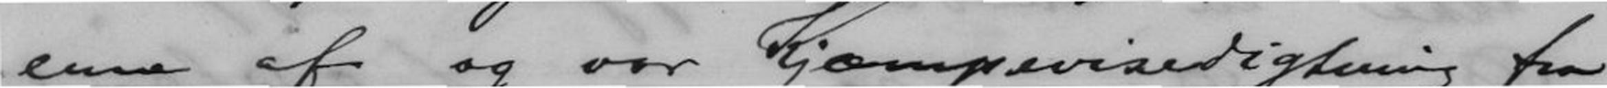erne af og vor Kjæmpevisedigtning fra
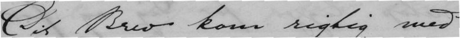Dit Brev kom rigtig med
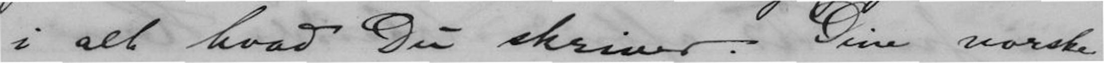i alt hvad Du skriver. Dine norske
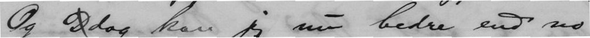Og D dog kan jeg nu bedre end no-
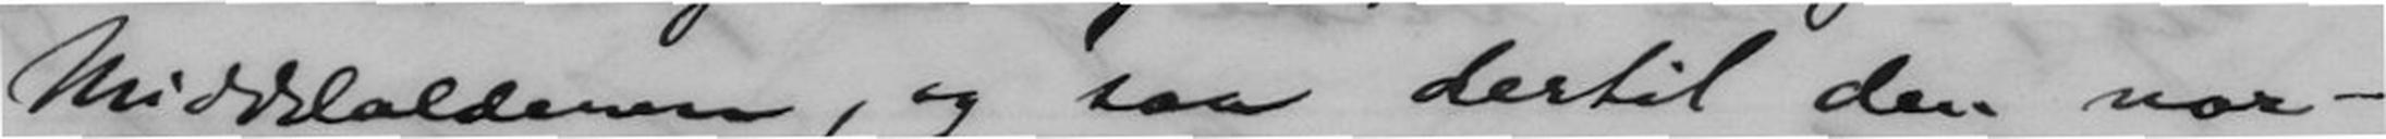Middelalderen, og saa dertil den nor-
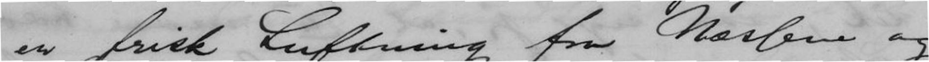en frisk Luftning fra Næssene og
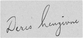Deres hengivne
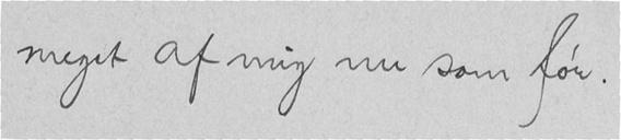meget af mig nu som før.
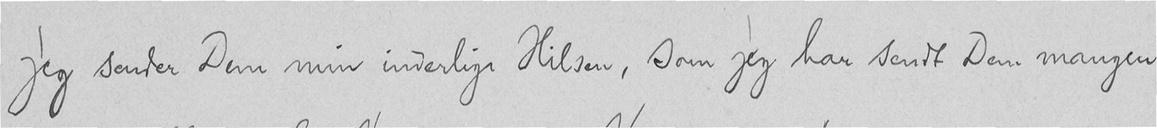jeg sender Dem min inderlige Hilsen, som jeg har sendt Dem mangen
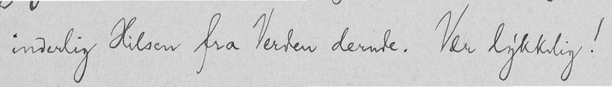inderlig Hilsen fra Verden derude. Vær lykkelig!
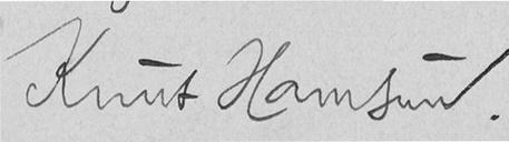Knut Hamsun.
